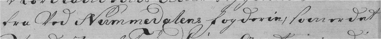fra Ved Nummedalens Fogderie, som er det
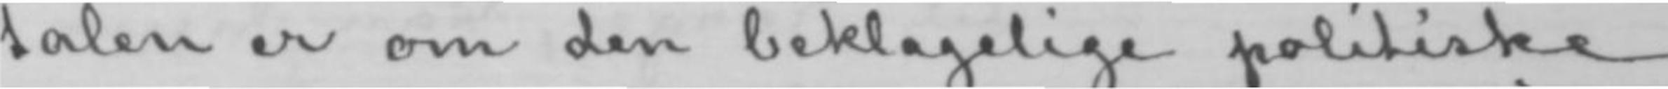talen er om den beklagelige politiske
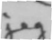jeg
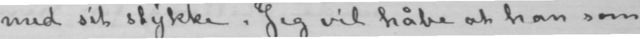med sit stykke. Jeg vil håbe at han som
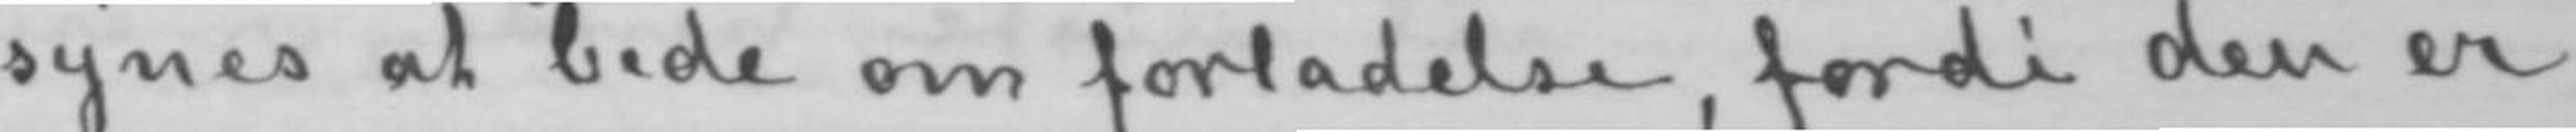synes at bede om forladelse, fordi den er
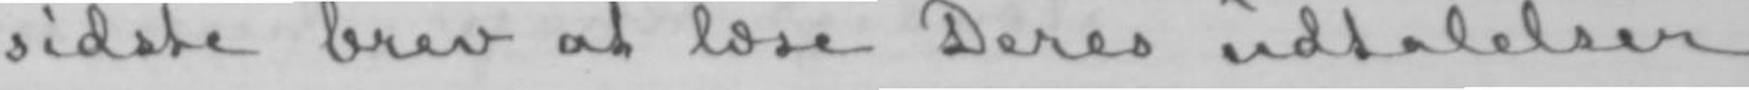sidste brev at læse Deres udtalelser
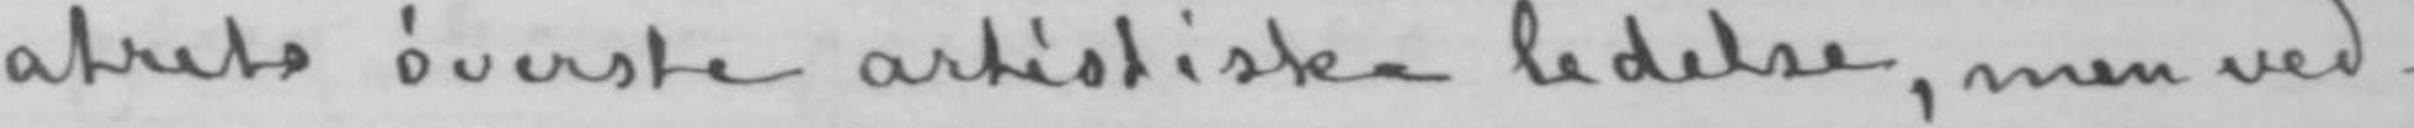atrets øverste artistiske ledelse, men ved-
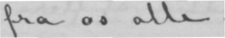fra os alle.
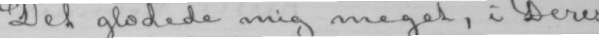Det glædede mig meget, i Deres
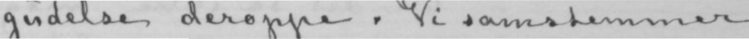gudelse deroppe. Vi samstemmer
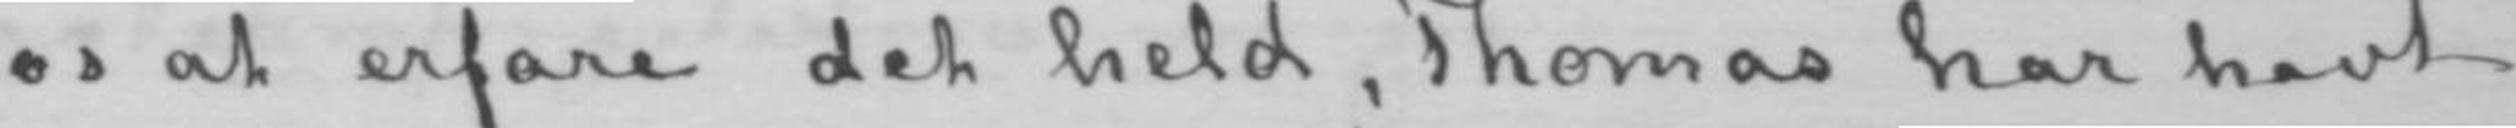os at erfare det held, Thomas har havt
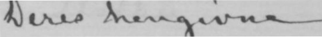Deres hengivne
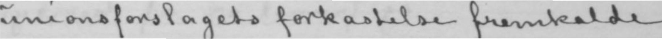unionsforslagets forkastelse fremkalde
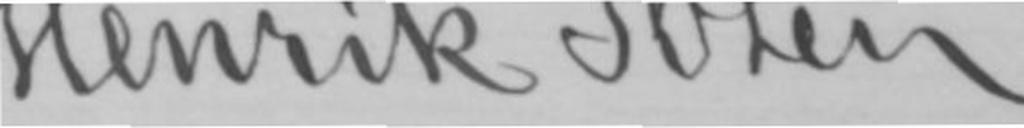Henrik Ibsen.
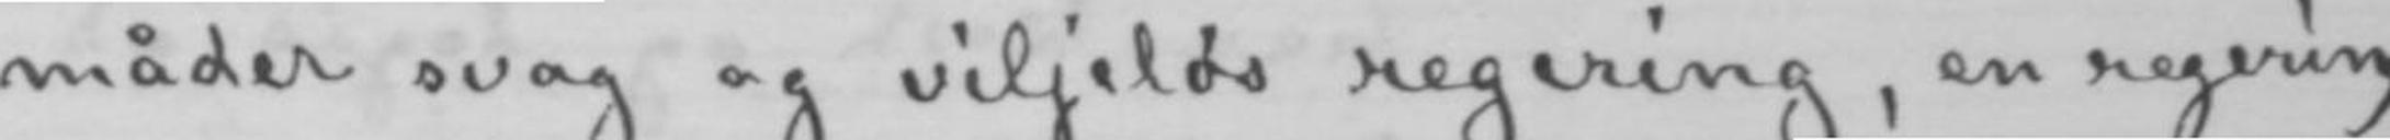måder svag og viljeløs regering, en regering,
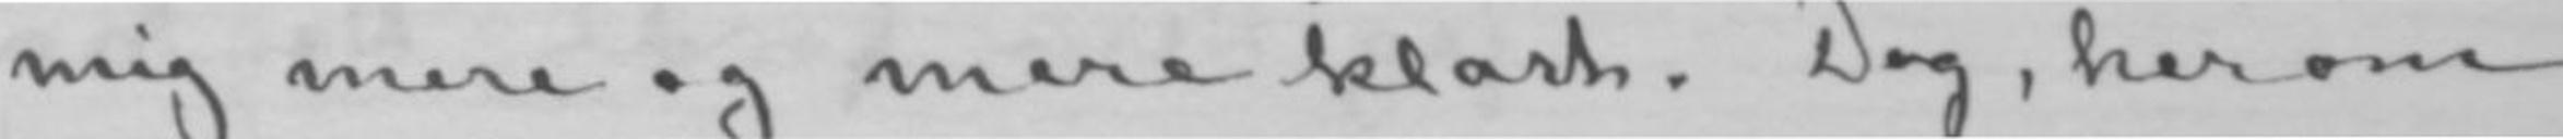mig mere og mere klart. Dog, herom
